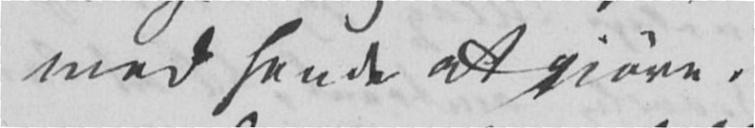med hende at gjøre.
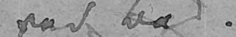ved Bad.
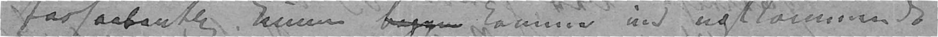forhaabentlig kunne begyn komme ind næstkommende
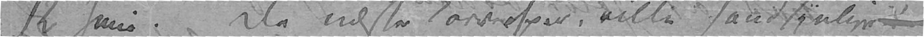12 Juni. De næste Korressper, ville sandsynlig[vis]
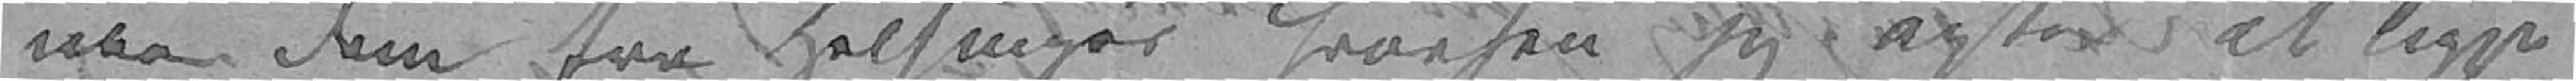naa Dem fra Helsingør hvorhen jeg agter at ligge
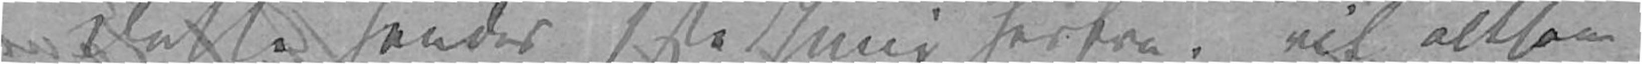Dette sendes 1ste Juni herfra, vil altsaa
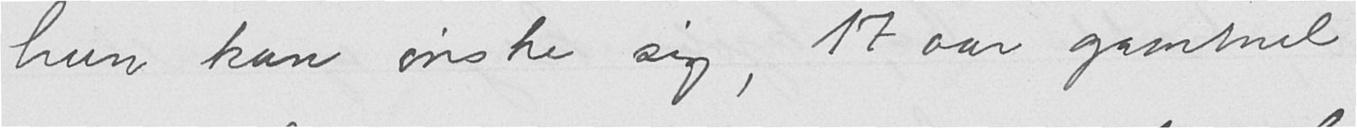hun kan ønske sig, 17 aar gammel
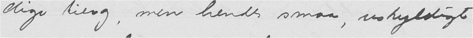dige ting, men hendes smaa, uskyldigt
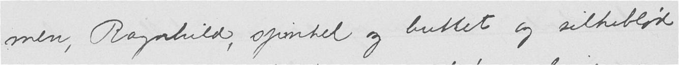men, Ragnhild, spinkel og buttet og silkeblød
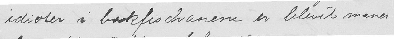idioter i backfischaarene er blevet maner-
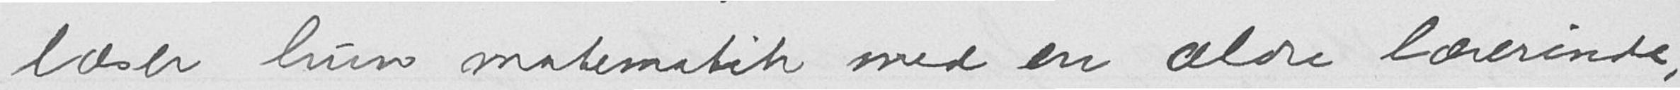læser hun matematik med en ældre lærerinde,
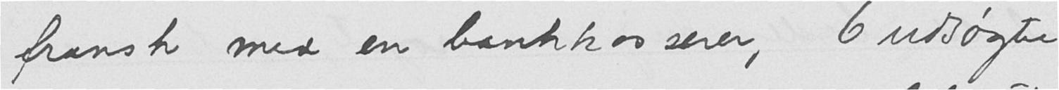fransk med en bankkasserer, 6 udsøgte
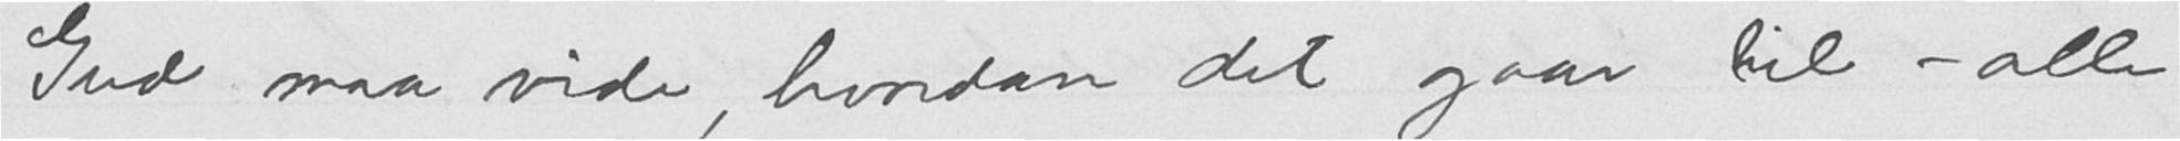Gud maa vide, hvordan det gaar til - alle
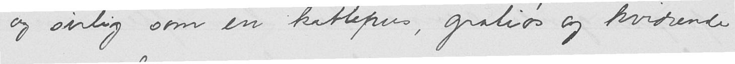og sirlig som en kattepus, gratiøs og kvidrende
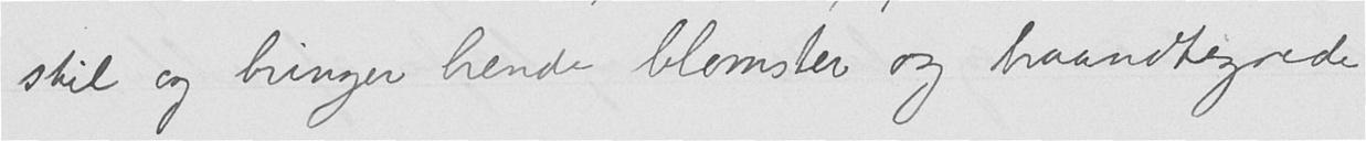stil og bringer hende blomster og haandtegnede
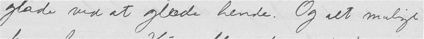glade ved at glæde hende. Og alt muligt
